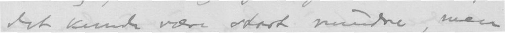det kunde være stort mindre, men
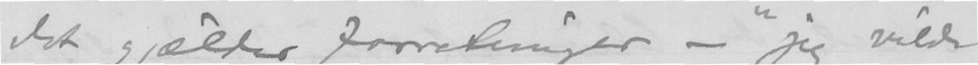det gjælder forretninger - "jeg vilde
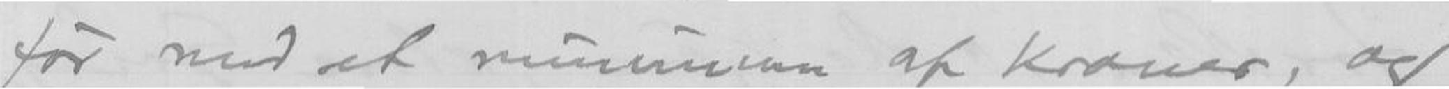før med et minimum af kroner, og
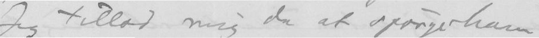Jeg tillod mig da at spørge ham
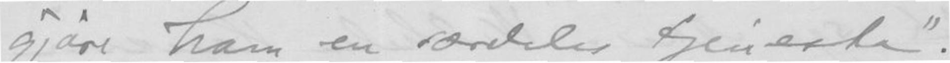gjøre ham en særdeles tjeneste".
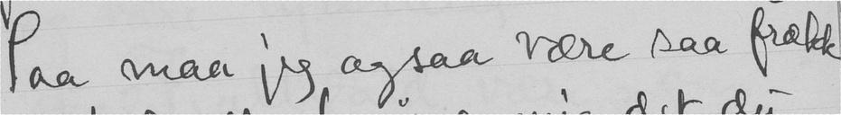Saa maa jeg ogsaa være saa frækk
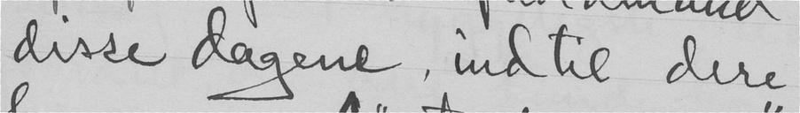disse dagene, indtil dere
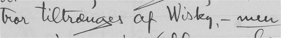tror tiltrænges af Wisky, - men
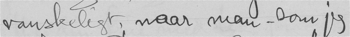vanskeligt, naar man - som jeg
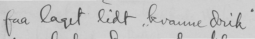faa laget lidt "kvanne drik"
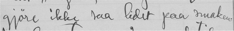gjøre ikke saa lidet paa smaken.
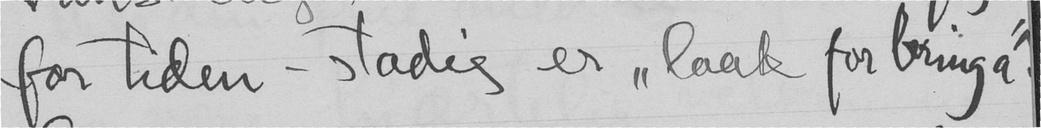for tiden - stadig er "laak for bringa".
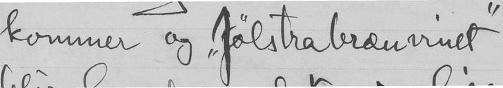kommer og "Jølstrabrænvinet"
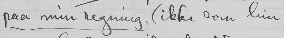paa min regning, (ikke som hin
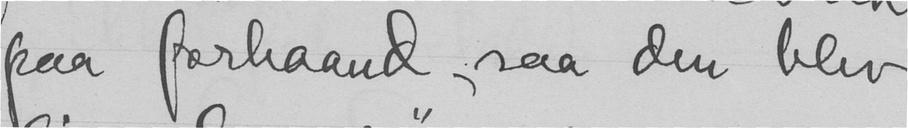paa forhaand, saa den blev
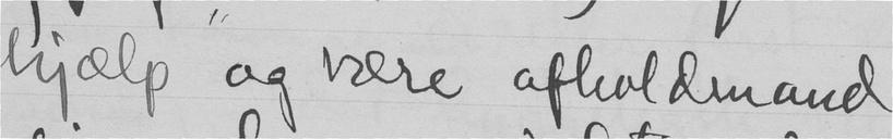hjælp" og være afholdmand
